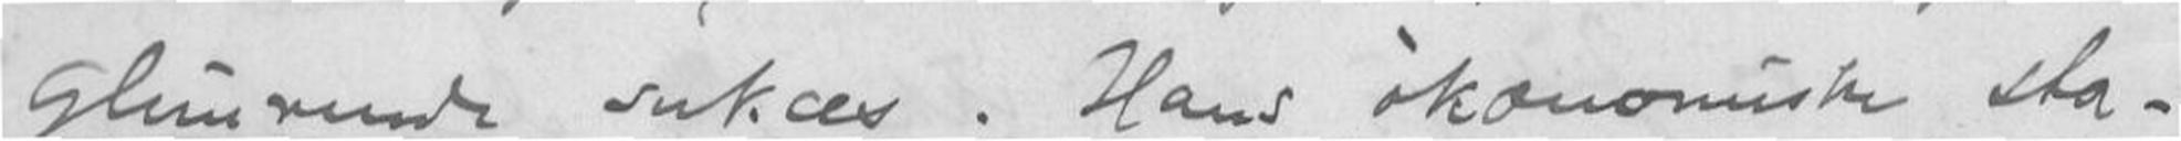glimrende sukces. Hans økonomiske sta-
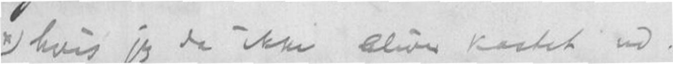x) hvis jeg da ikke bliver kastet ud.
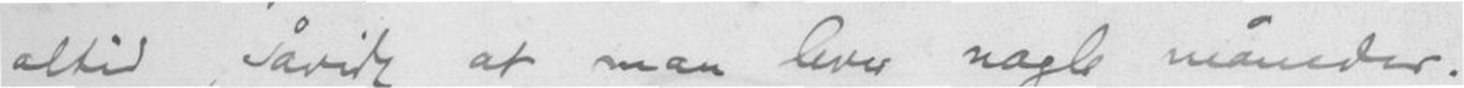altid, såvidt at man lever nogle måneder.
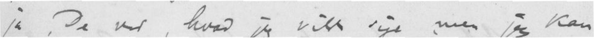ja, De ved, hvad jeg vilde sige men jeg kan
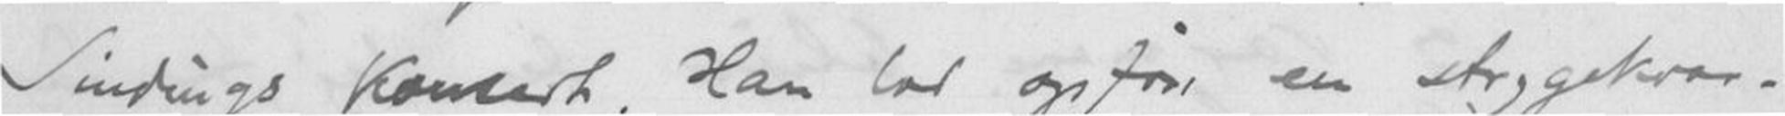Sindings Konsert. Han lad opføre en strygekvar-
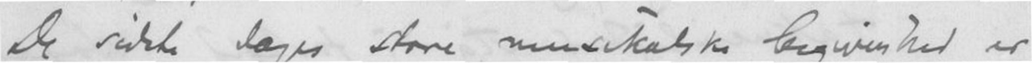De sidste dages store musikalske begivenhed er
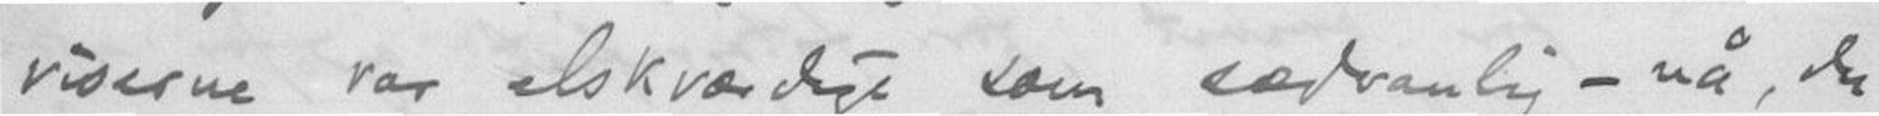viserne var elskværdige som sædvanlig - nå, du
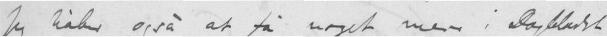Jeg håber også at få noget mere i Dagbladet
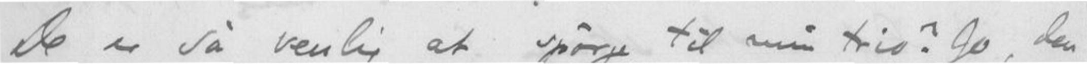De er så venlig at spørge til min trio? Jo, den
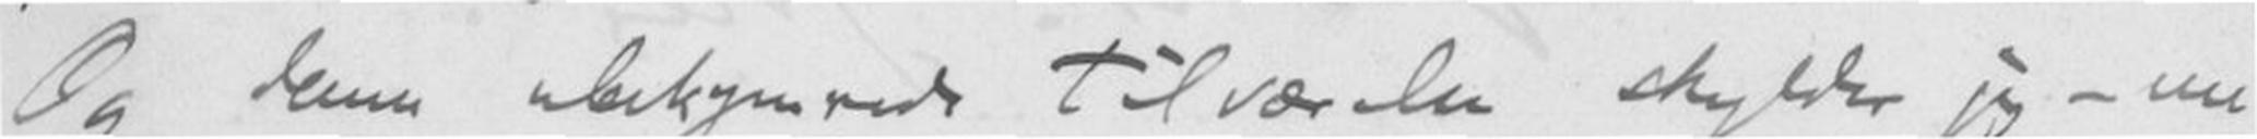Og denne ubekymrende tilværelsen skylder jeg - nu
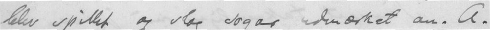blev spillet og slog sogar udmærket an, A-
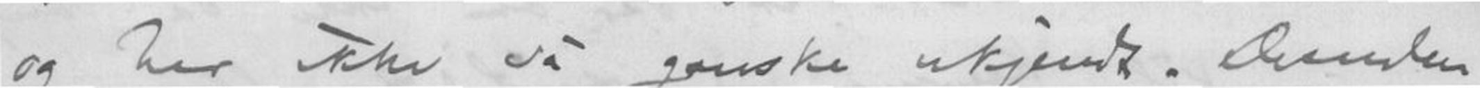og her ikke så ganske ukjendt. Desuden
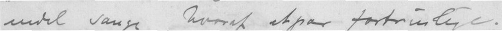endel sange, hvoraf et par fortinlige
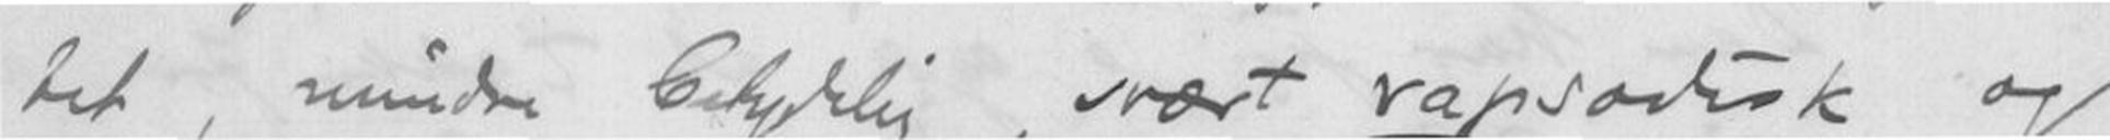tet, mindre betydelig, svært rapsodisk og
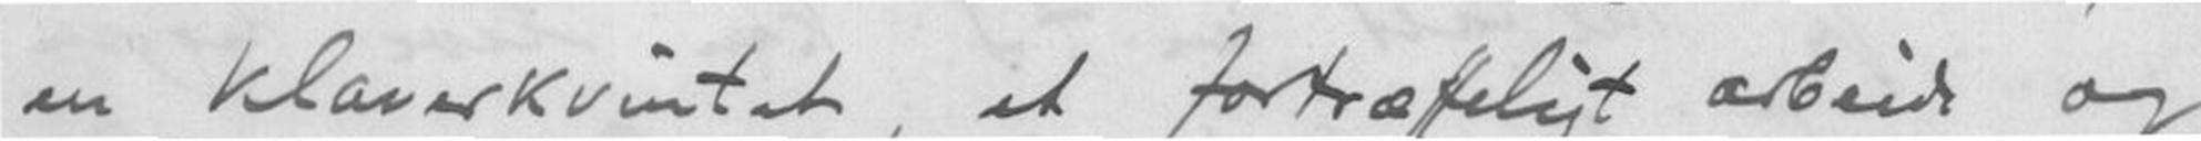en klaverkvintet, et fortræffeligt arbeide og
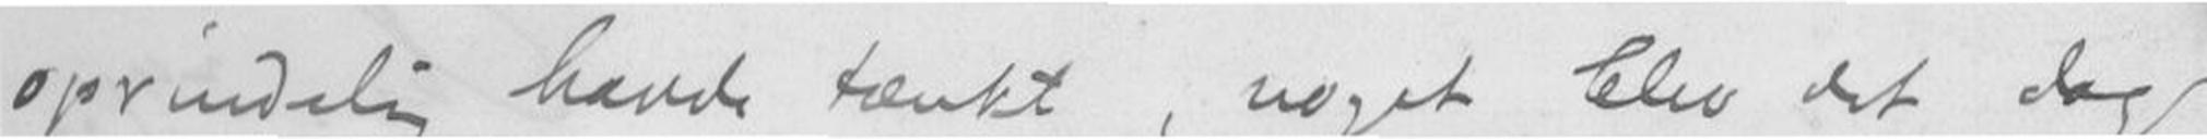oprindelig havde tænkt, noget blev det dog
